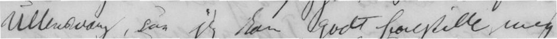Ullensvang, saa jeg kan godt forestille mig
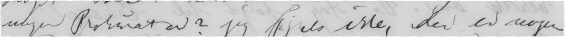nogen Prokurator? jeg synes ikke, der er nogen
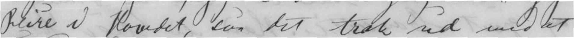Reise i Hovedet, som det trak ud med at
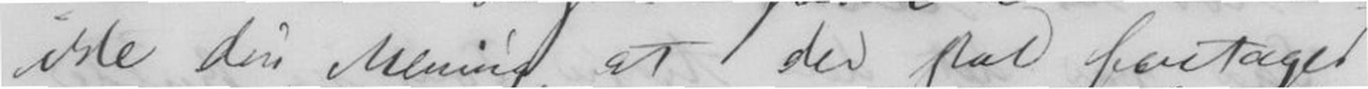ikke din Mening, at der skal foretages
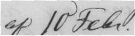af 10 Feb.
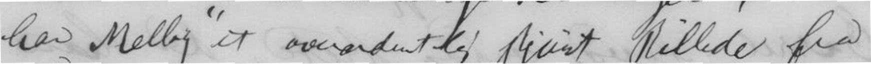har Melby et overordentlig skjønt Billede fra
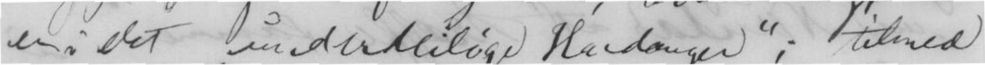er i det underblilige Hardanger; tilmed
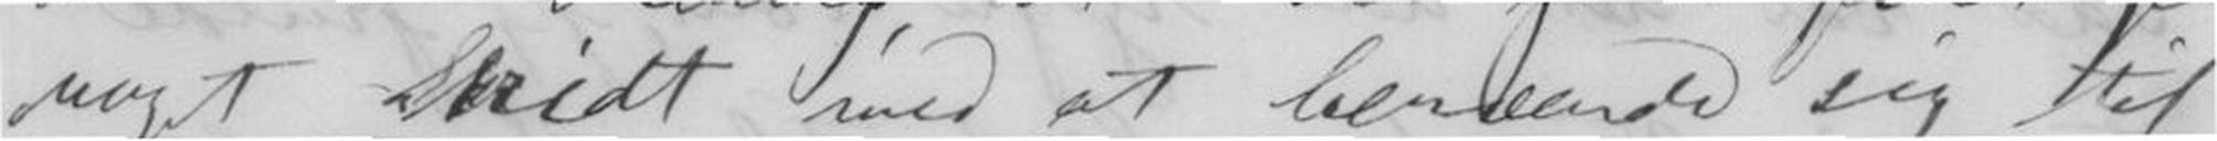noget skridt med at henvende sig til
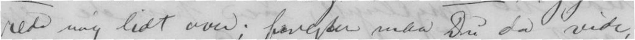rede mig lidt over; forresten maa Du da vide,
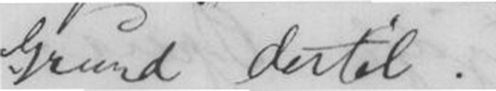Grund dertil.
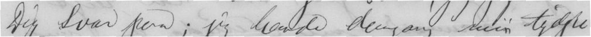Dig Svar paa; jeg havde dengang min tydske
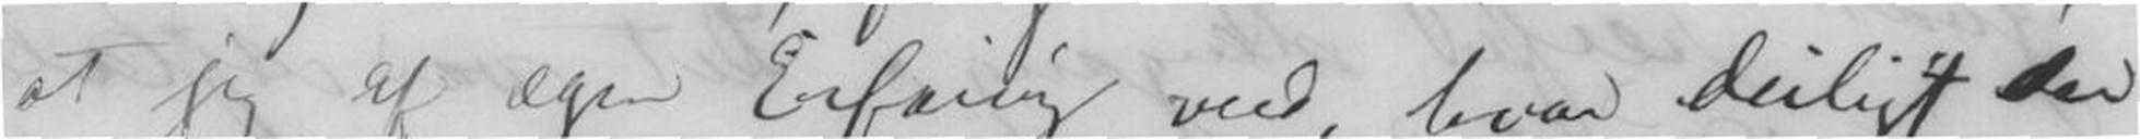at jeg af egen Erfaring ved, hvor deiligt der
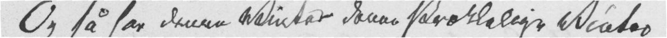Og så har denne Vinter – denne skrækkelige Vinter
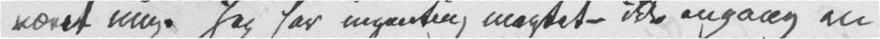været mig. Jeg har ingenting magtet – ikke engang en
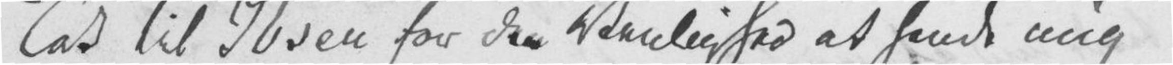Tak til Ibsen for den Venlighed at sende mig
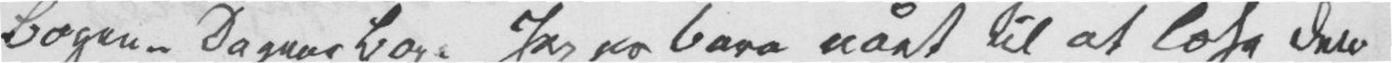Bogen – Dagens Bog. Jeg er bare nået til at læse den
